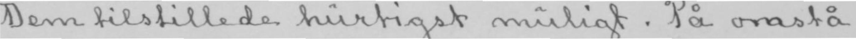Dem tilstillede hurtigst muligt. På onmstå-
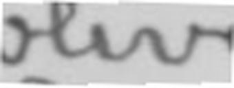blive
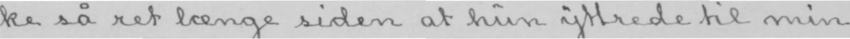ke så ret længe siden at hun yttrede til min
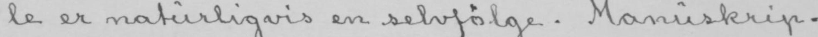le er naturligvis en selvfølge. Manuskrip-
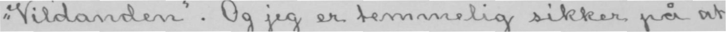«Vildanden». Og jeg er temmelig sikker på at
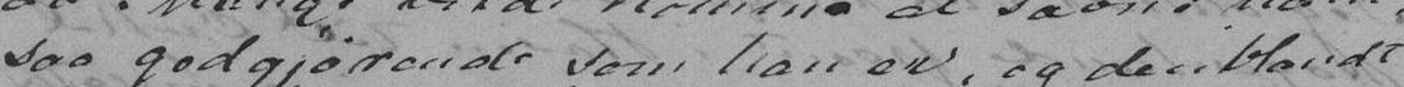saa godgjørende som han er, og deriblandt
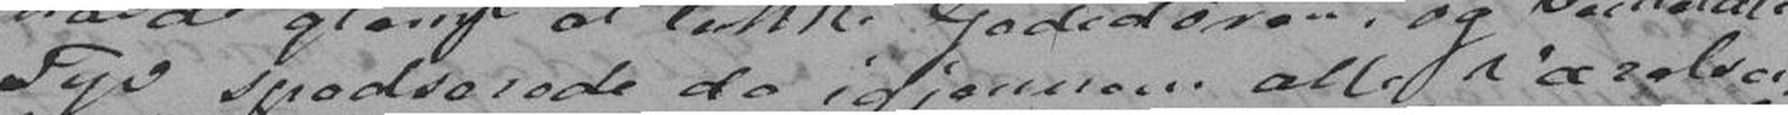Tyv spadserede da igjennem alle Værelser,
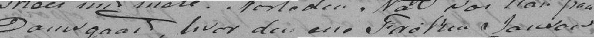Damsgaard hvor den ene Frøken Janson
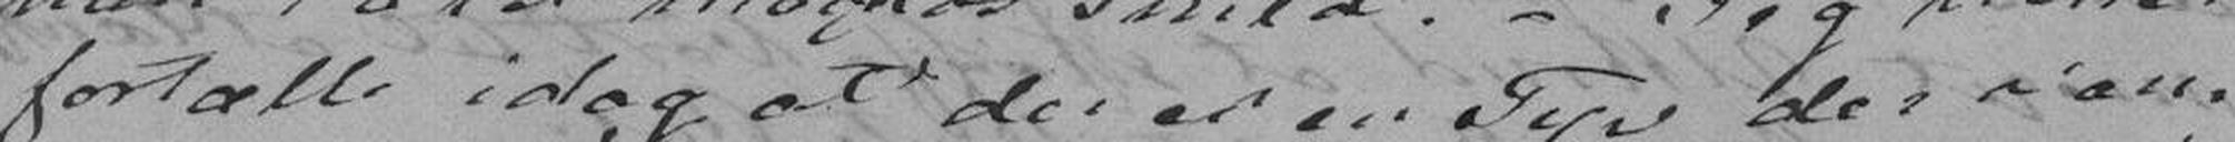fortælle idag at der er en Tyv der van-
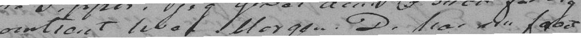omtrent hver Morgen. De har nu faaet
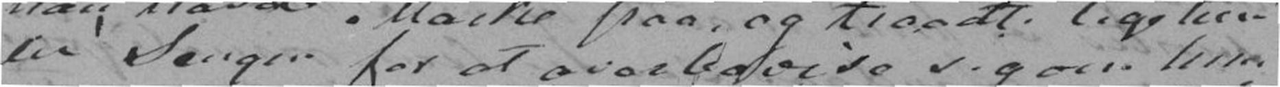til Sengen for at overbevise sig om hun
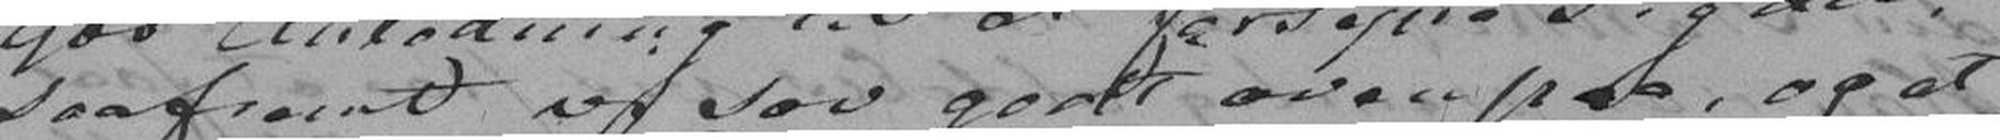saafremt vi sov godt ovenpaa, og at
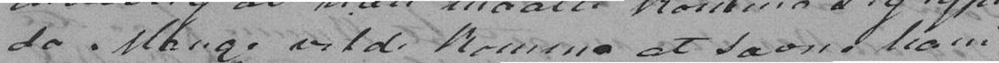da Mange vilde komme at savne ham,
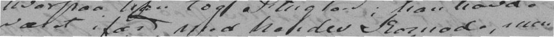været ifærd med hendes Komode, men
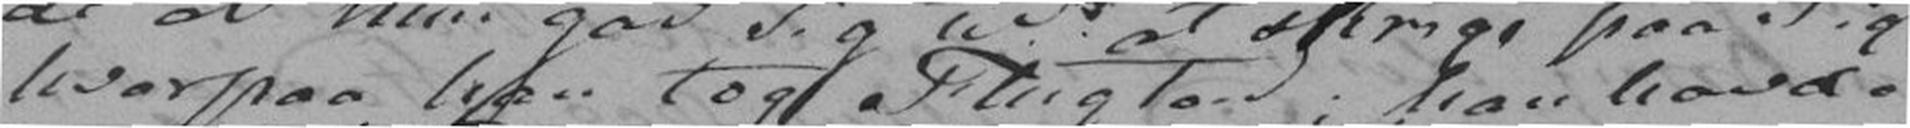hvorpaa han tog Flugten; han havde
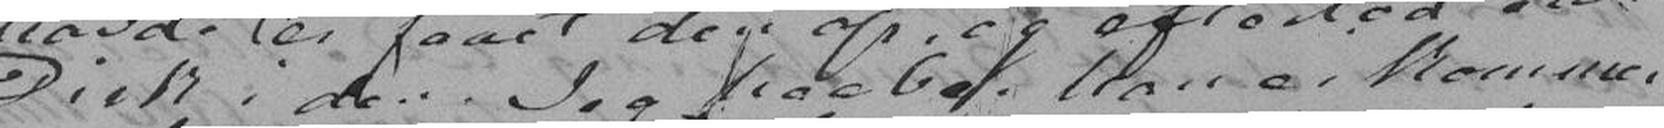Dirk i den. Jeg haaber han ei kommer
